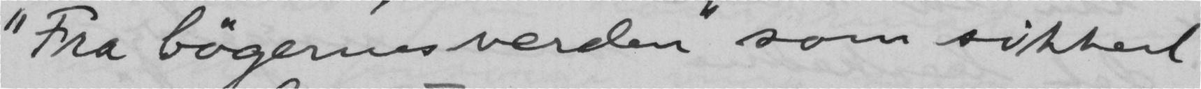"Fra bøgernes verden" som sikkert
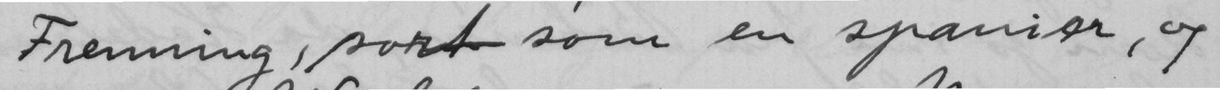Frenning, sort som en spanier, og
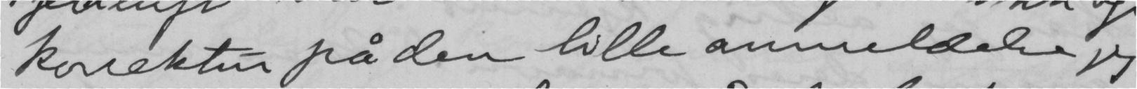korrektur på den lille anmeldene jeg
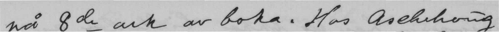på 8de ark av boka. Hos Aschehoug
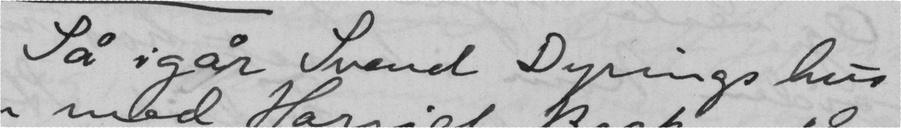Så igår Svend Dyrings hus
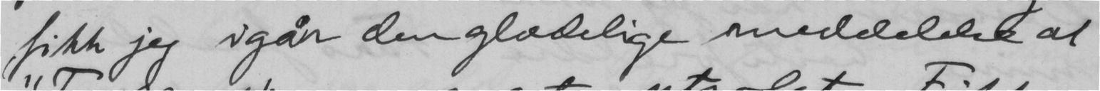fikk jeg igår den glædelige meddelelse at
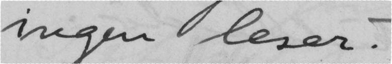ingen leser.
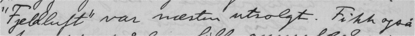"Fjeldluft" var næsten utsolgt. Fikk også
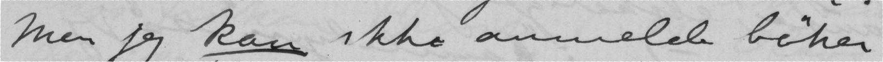Men jeg kan ikke ammelde bøker
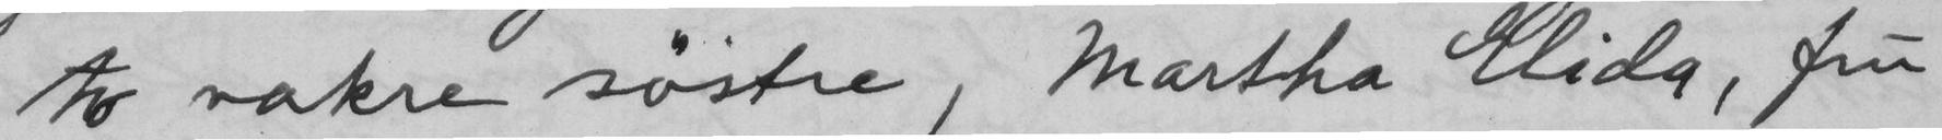to vakre søstre, Martha Elida, fru
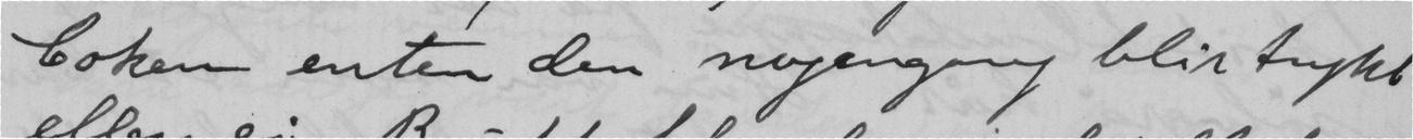boken enten den nogengang blir trykt
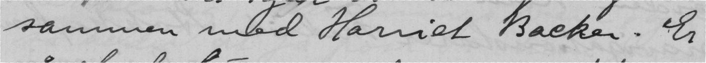sammen med Harriet Backer. Er
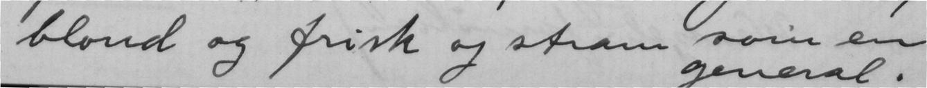blond og frisk og stram som en
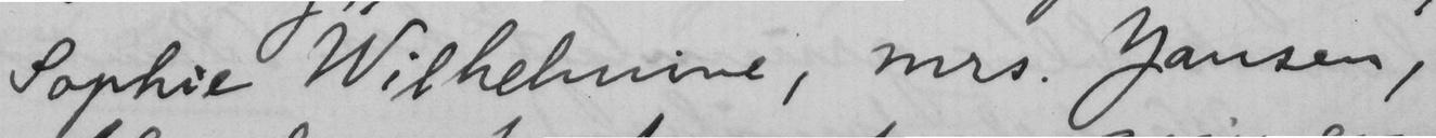Sophie Wilhelmine, mrs. Jansen,
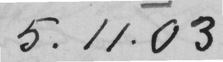5.11.03
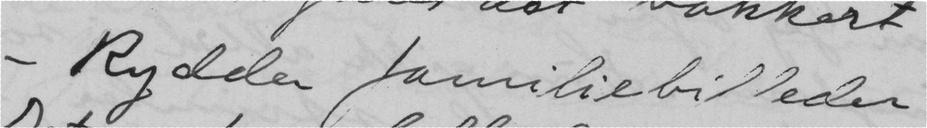– Rydder familiebilleder
